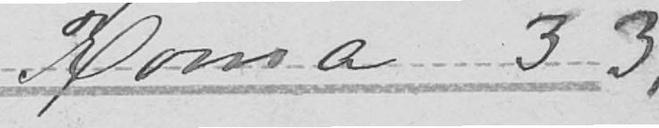Roma 33
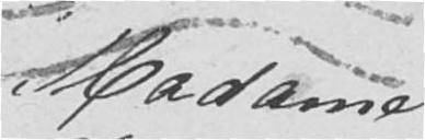Madame
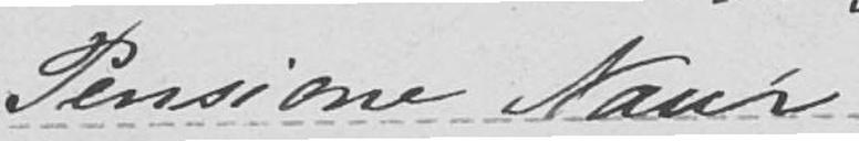Pensione Naur
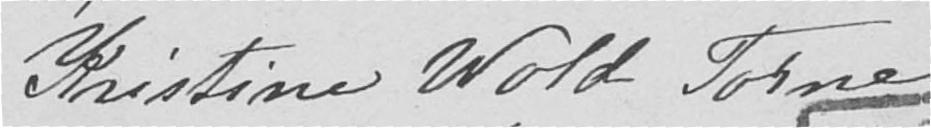Kristine Wold Torne
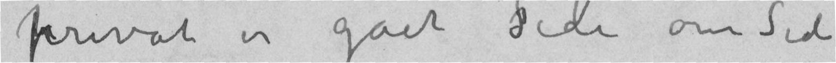privat er gået Side om Side
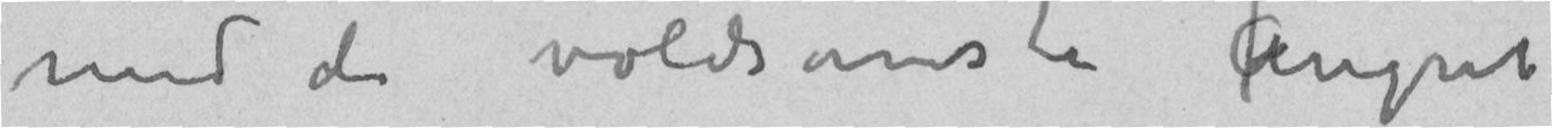med de voldsomste Angreb
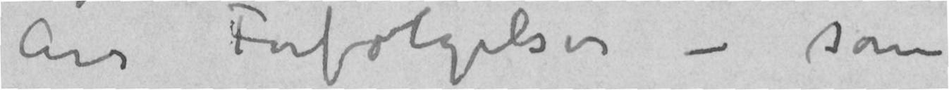års Forfølgelser – som
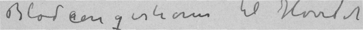Blodcongestioner til Hovedet
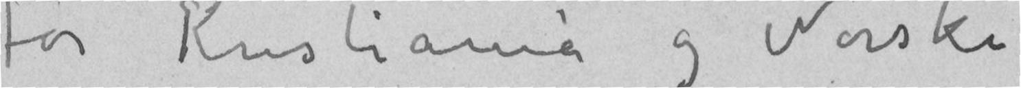for Kristiania og Norske
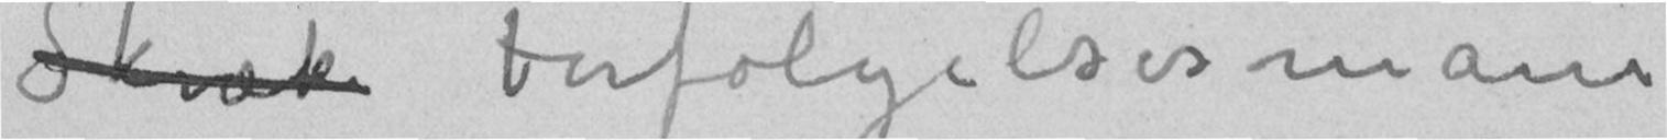Skræk Forfølgelsesmani
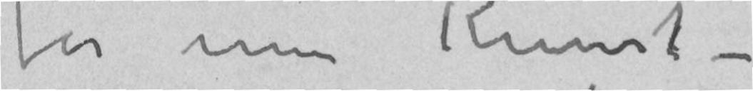på min Kunst –
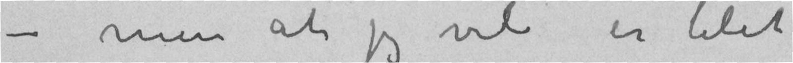– men at jeg vel er blit
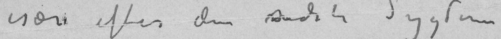især efter den sidste Sygdom
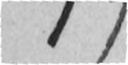1)
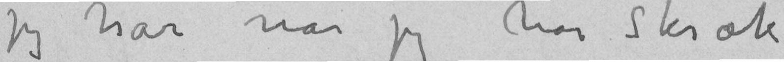jeg har når jeg har Skræk
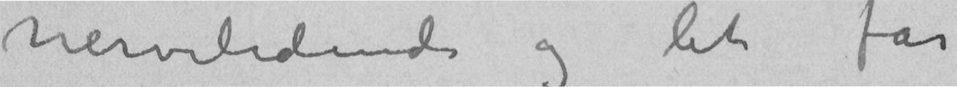nervelidende og let får
